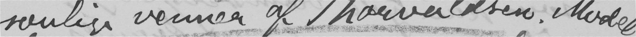sonlige venner af Thorvaldsen. Model-
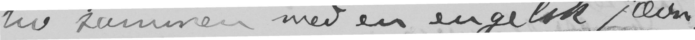liv sammen med en engelsk jævn-
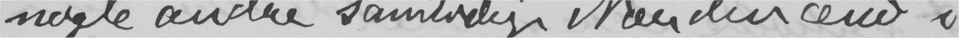nogle andre samtidige Nordmænd i
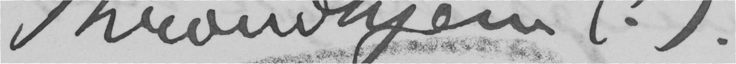Throndhjem (!).
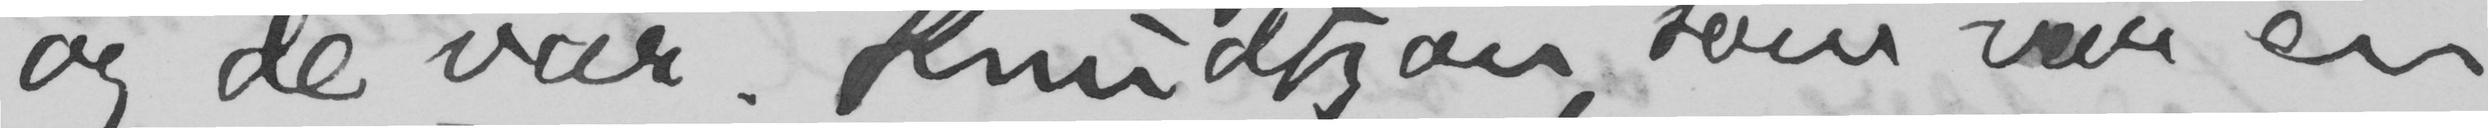og de var. Knudtzon, som var en
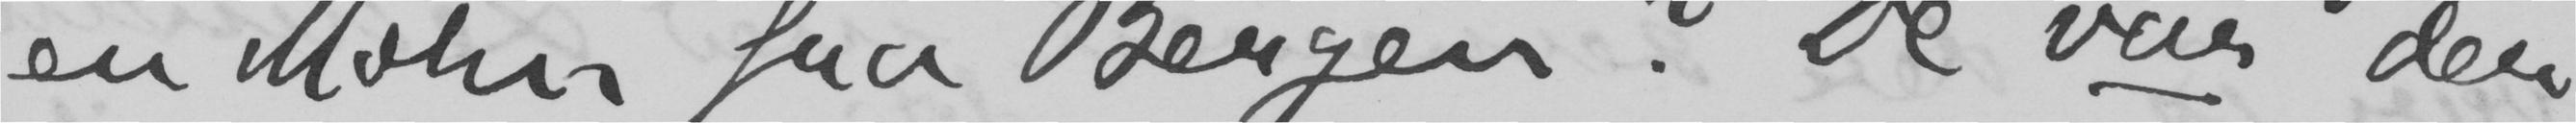en Mohn fra Bergen? De var der
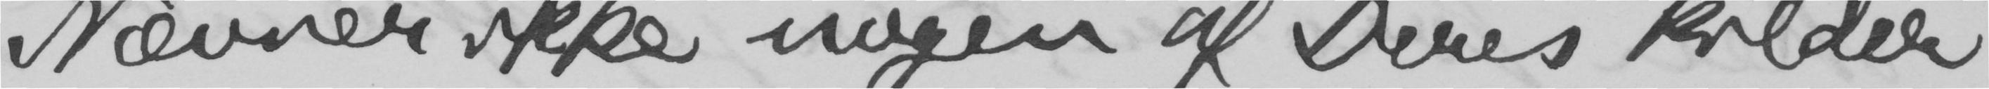Nævner ikke nogen af Deres kilder
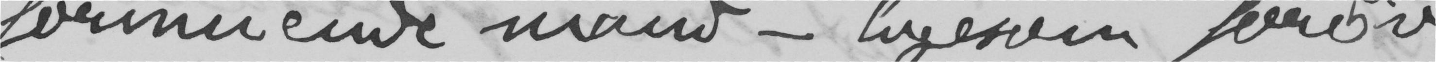formuende mand – ligesom forøv-
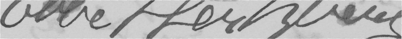Ebbe Hertzberg
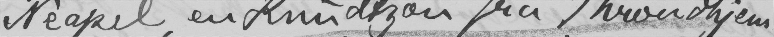Neapel, en Knudtzon fra Throndhjem,
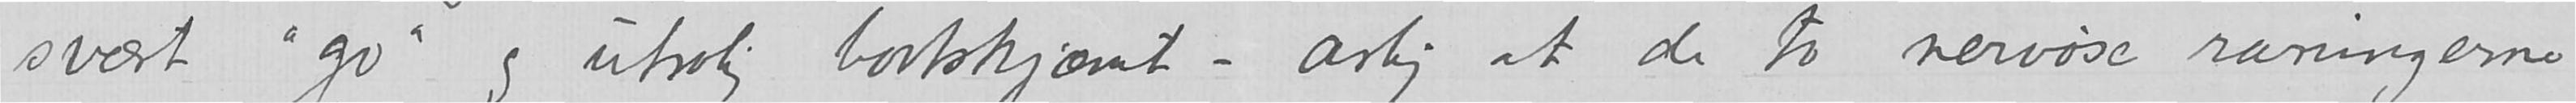svært «go» og utrolig bortskjæmt – artig at de to nervøse raringerne
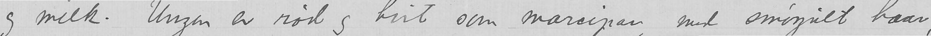og melk. Ungen er rød og hvit som marsipan, med smørgult haar,
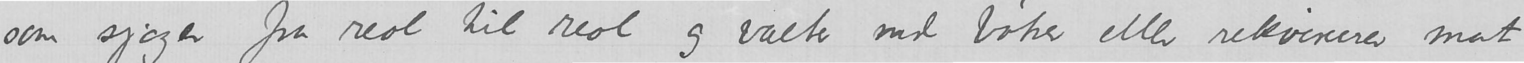som sjoger fra reol til reol og vælter ned bøker eller rekvirerer mat
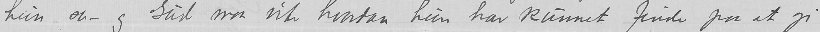hun sa og Gud maa vite hvordan hun har kunnet finde paa at gi
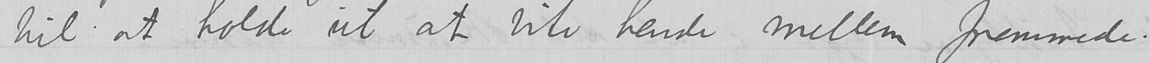til at holde ut at vite hende mellem fremmede.
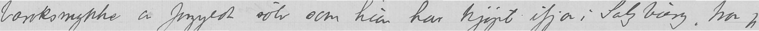baroksmykke av forgyldt sølv som hun har kjøpt ifjor i Salzburg, tror jeg
